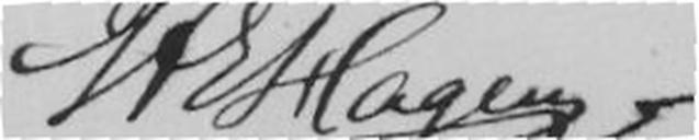S A E Hagen
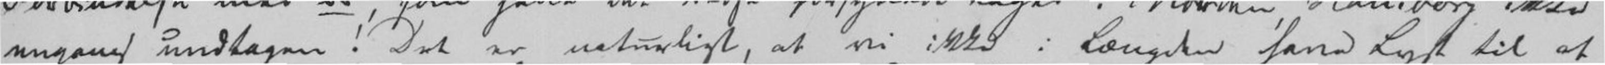engang undtagen! Det er naturligt, at vi ikke i Længden have Lyst til at
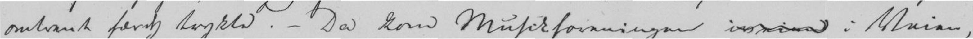omtrent færdig trygte. - Da kom Musikforeningen iveien i Veien,
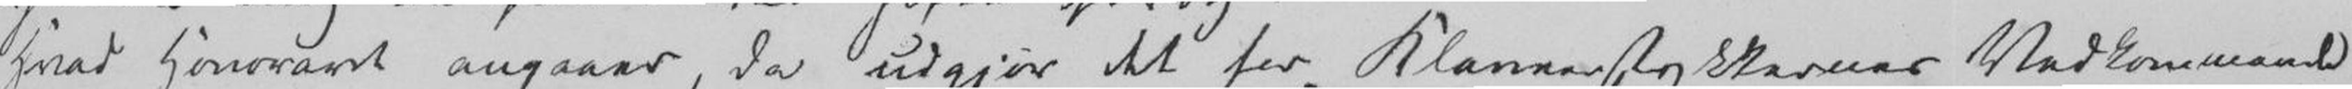Hvad Honoraret angaar, da udgjør det for Klaverstykkernes Vedkommende
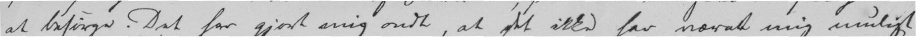at besørge. Det har gjort mig ondt, at det ikke har været mig muligt
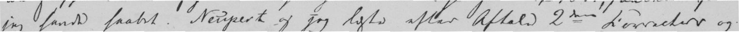jeg havde haabet. Neupert og jeg læste efter Aftale 2den Correctur og
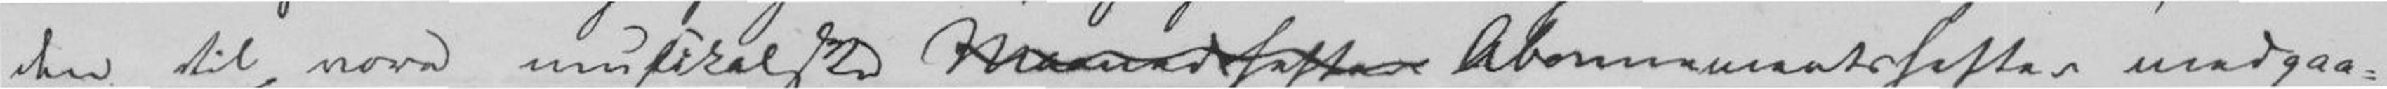den til vore musikalske Maanedshefter Abonnementshefter medgaa-
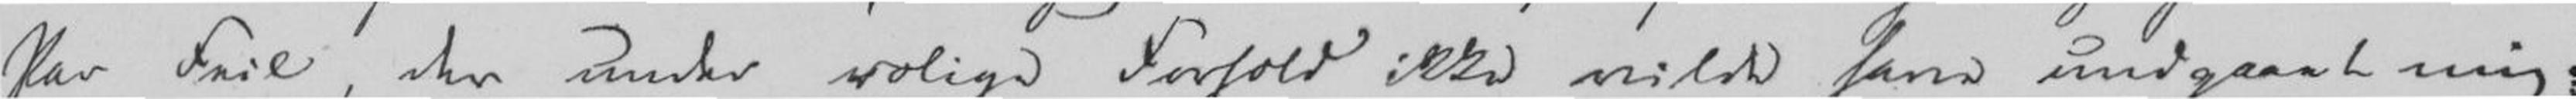Par Feil, der under rolige Forhold ikke vilde have undgaaet mig;
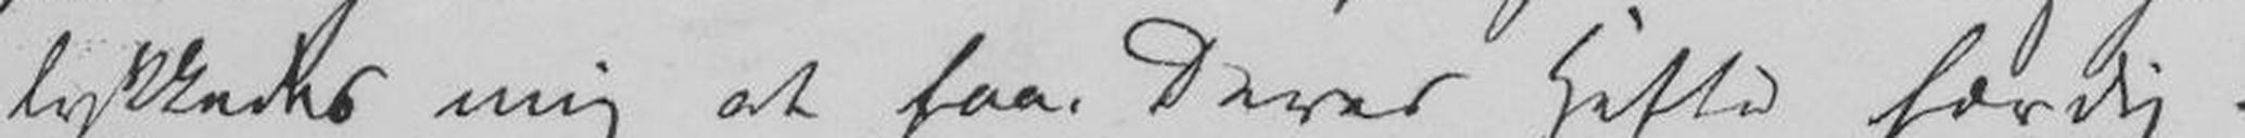lykkedes mig at faa Deres Hefte færdigt.
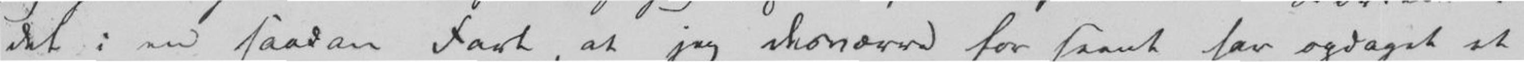det i en sadan Fart, at jeg desværre for seent har opdaget et
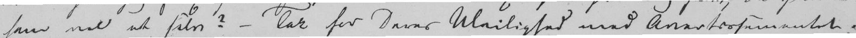ham vel et selv? - Tak for deres Uleilighed med Avertessementet;
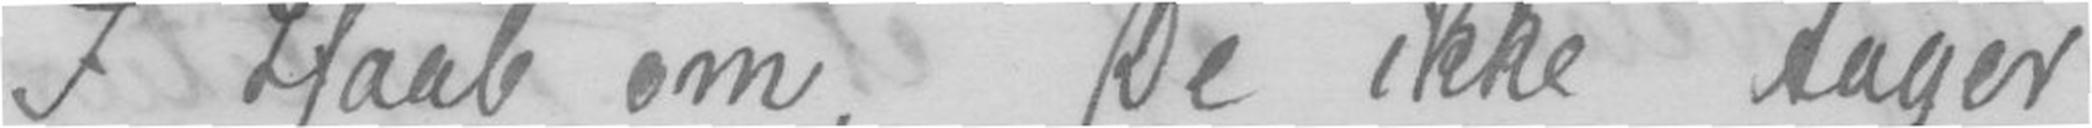I Haab om, De ikke tager
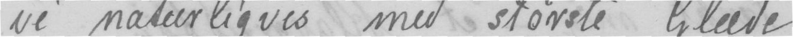vi naturligvis med største Glæde
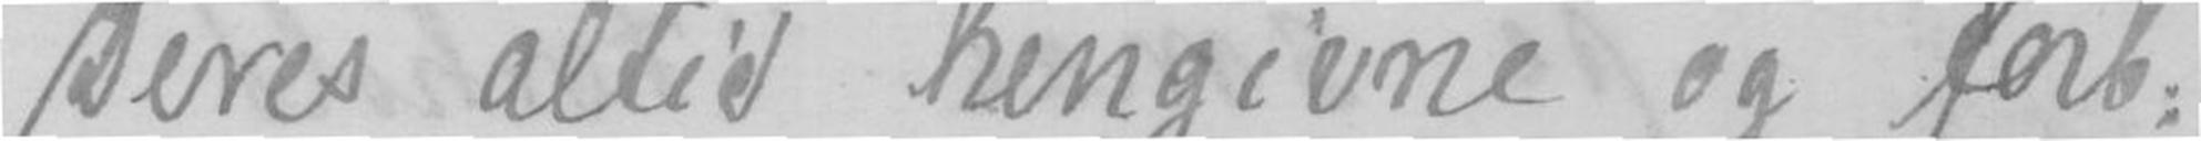Deres altid hengivne og forb.
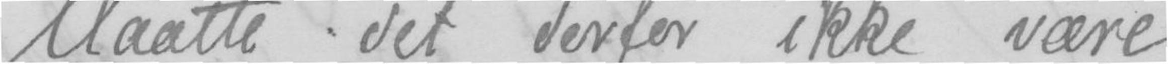Maatte det derfor ikke være
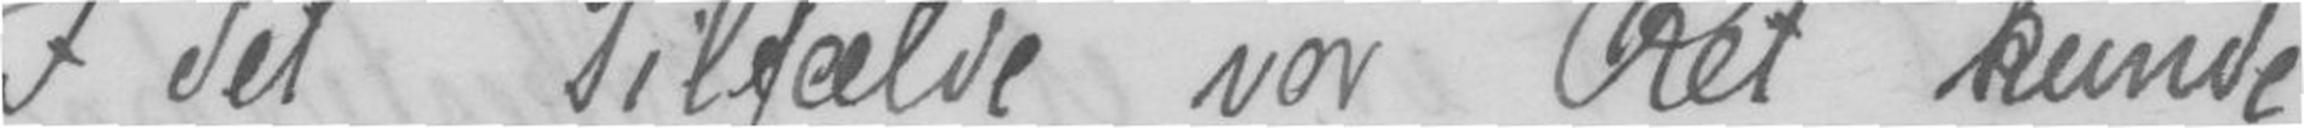I det Tilfælde vor Ret kunde
